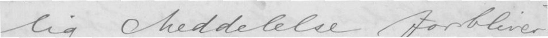lig Meddelelse forbliver
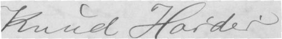Knud Harder
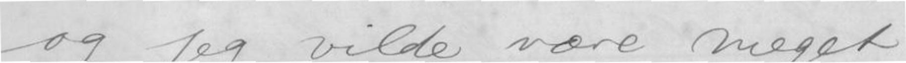og jeg vilde være meget
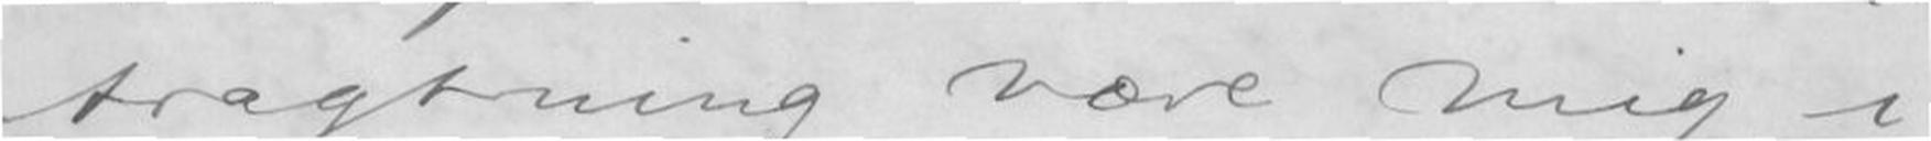tragtning være mig i
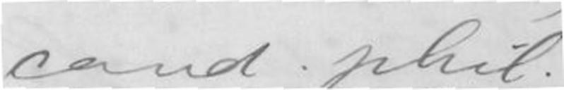cand. phil.
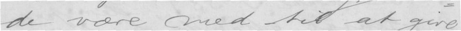de være med til at give
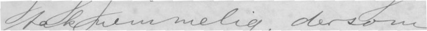taknemmelig, dersom
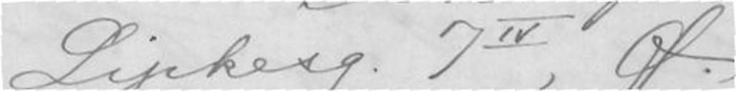Lipkesg. 7IV, Ø.
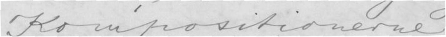Kompositionerne
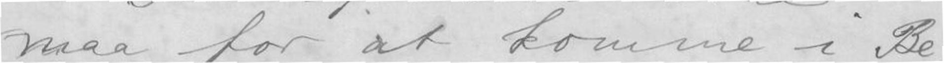maa for at komme i Be-
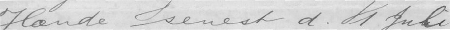Hænde senest d. 1 Juli
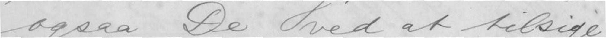ogsaa De, ved at tilsige
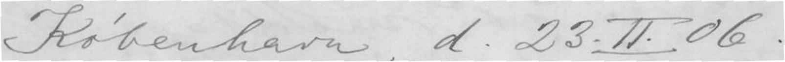København, d. 23.II.06.
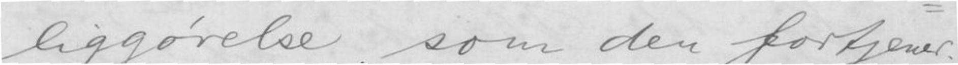liggørelse, som den fortjener.
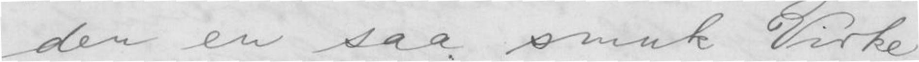den en saa smuk Virke
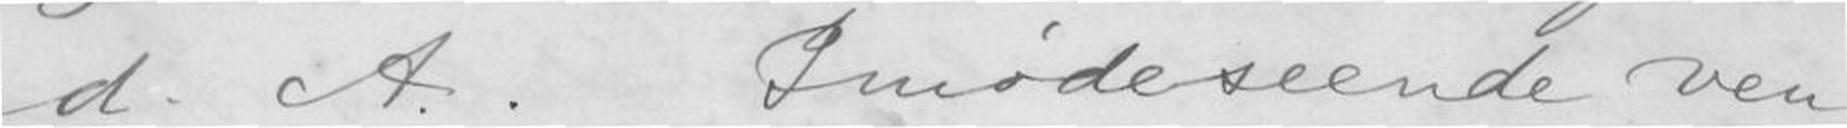d. A. Imødeseende ven-
